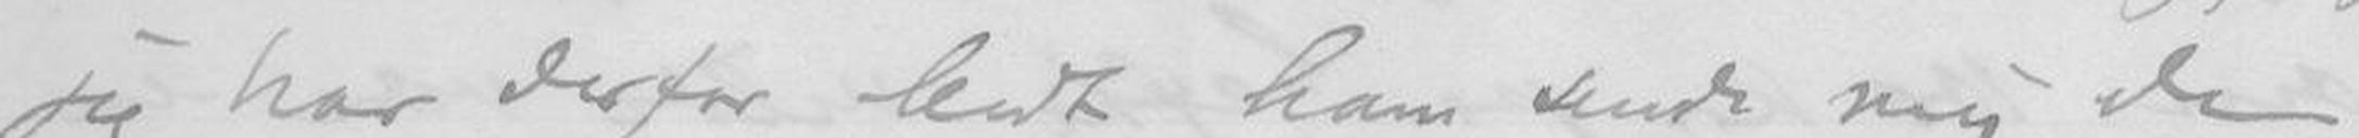jeg har derfor bedt ham sende mig de
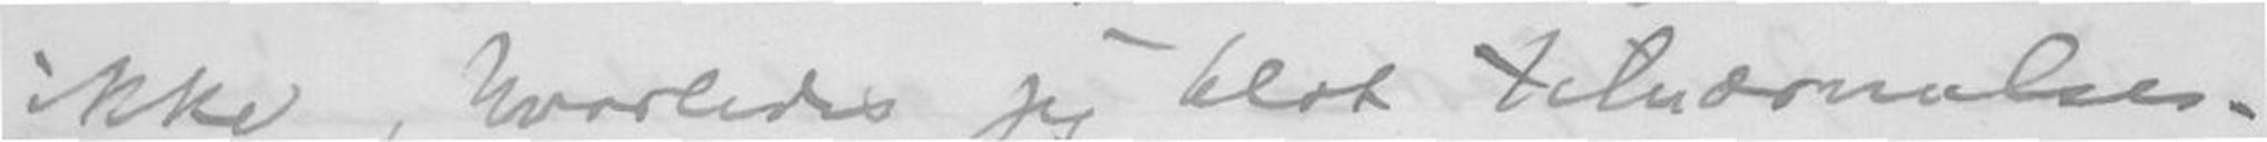ikke, hvorledes jeg blot tilnærmelses-
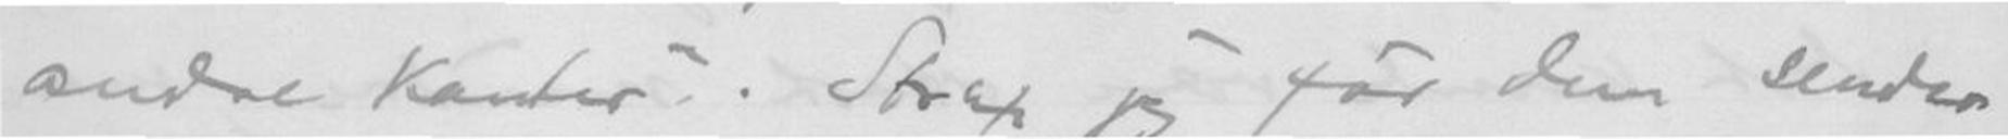andre kanter". Strax jeg får dem sender
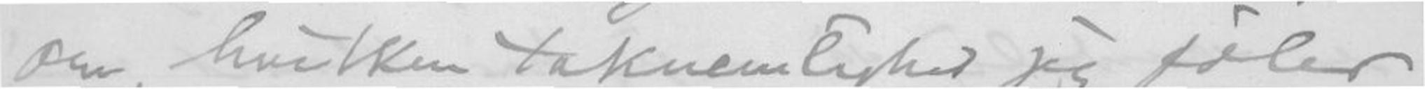om, hvilken taknemlighed jeg føler
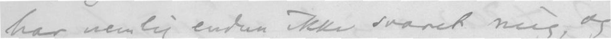har nemlig endnu ikke svaret mig, og
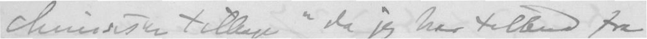chinesiske tilbage "da jeg har tilbud fra
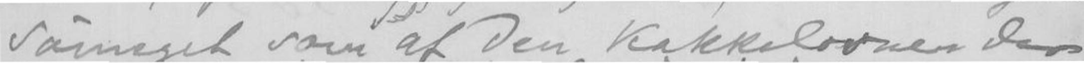såmeget som af Den kakkelovne der
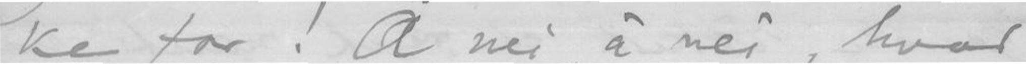ke for! Å nei, å nei, hvad
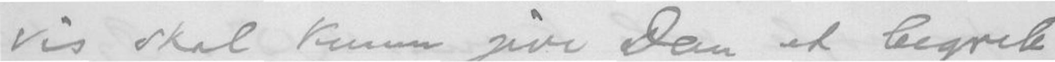vis skal kunne give Dem et begreb
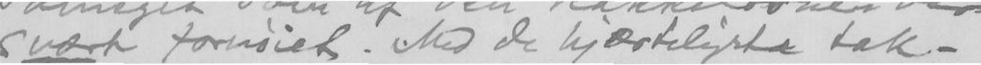svært fornøiet. Med de hjærteligste tak-
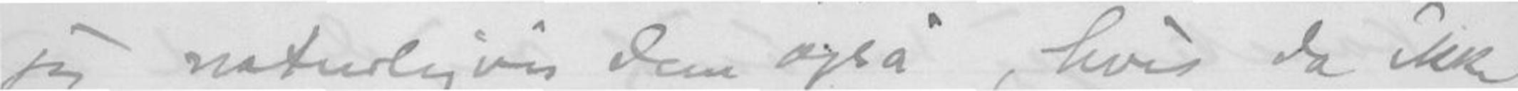jeg naturligvis dem også, hvis da ikke
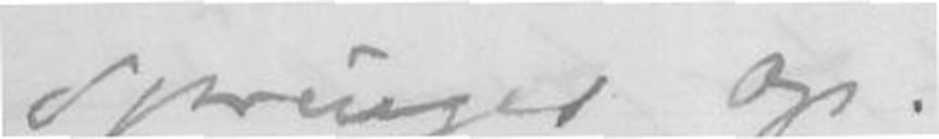springer op.
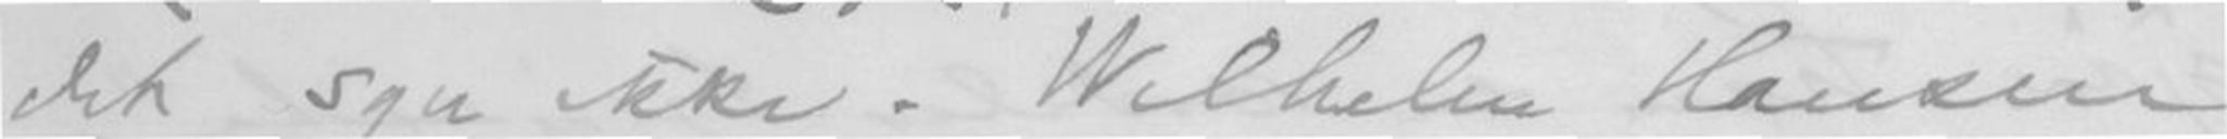det sju ikke. Wilhelm Hansen
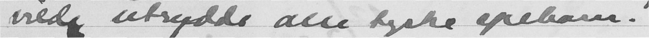vilde utrydde alle tyske spebarn!
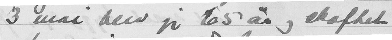3 mai blev jeg 65 år og skoftet
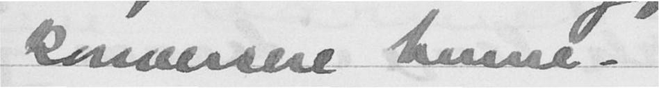konessere henne.
